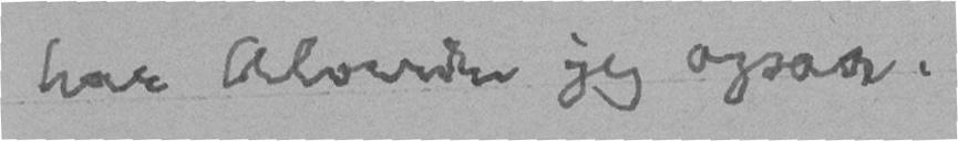har Alverden jeg ogsaa.
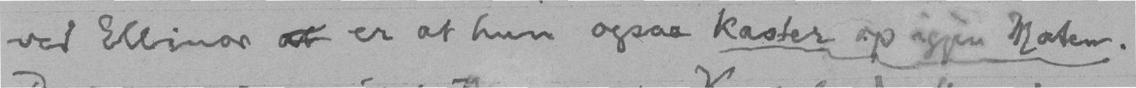ved Ellinor at er at hun ogsaa kaster op igjen Maten.
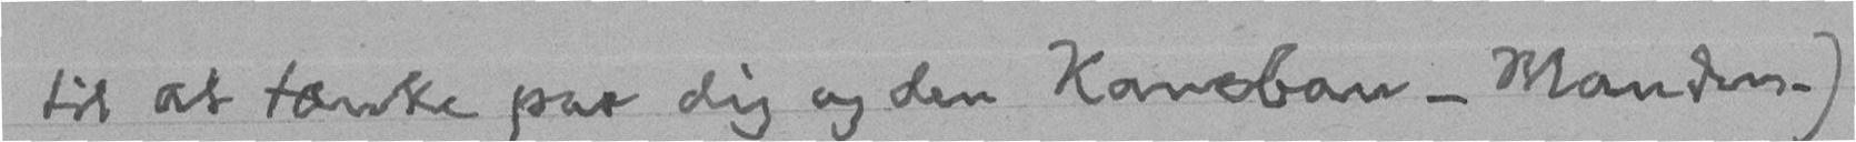til at tænke paa dig og den Kamban-Manden.)
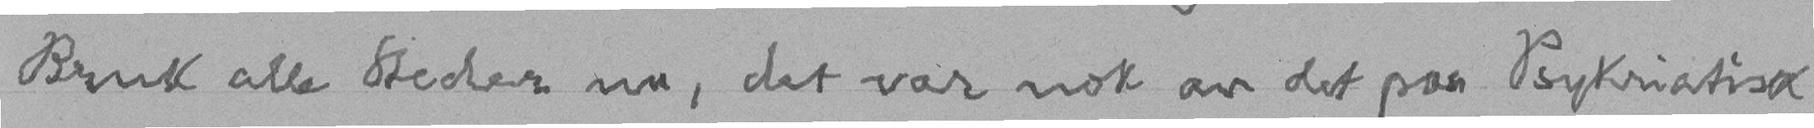Bruk alle Steder nu, det var nok av det paa Psykriatisk
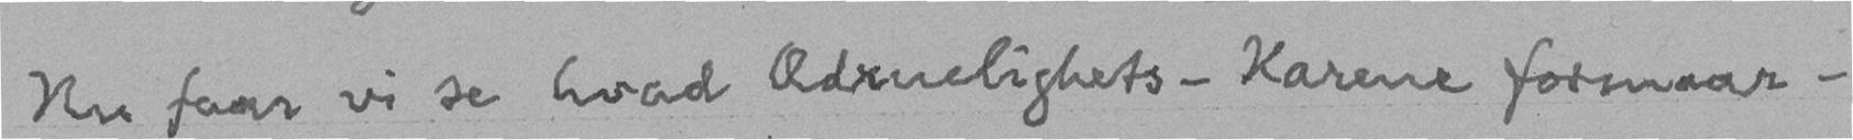Nu faar vi se hvad Ædruelighets-Karene formaar -
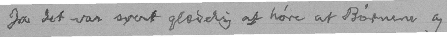Ja det var svert glædelig at høre at Børnene og
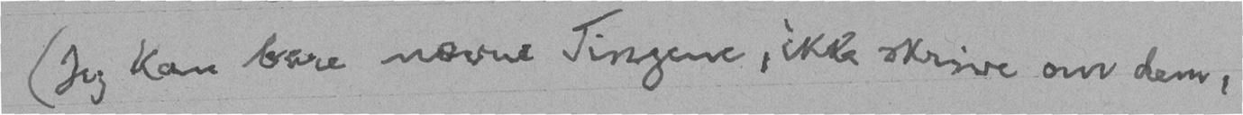(Jeg kan bare nævne Tingene, ikke skrive om dem,
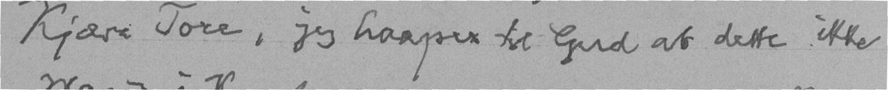Kjære Tore, jeg haaper til Gud at dette ikke
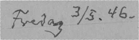Fredag 3/5. 46.
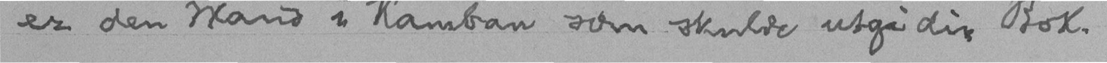er den Mand i Kamban som skulde utgi din Bok.
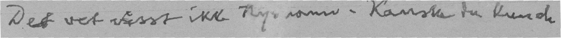Det vet visst ikke Nymann. Kanske du kunde
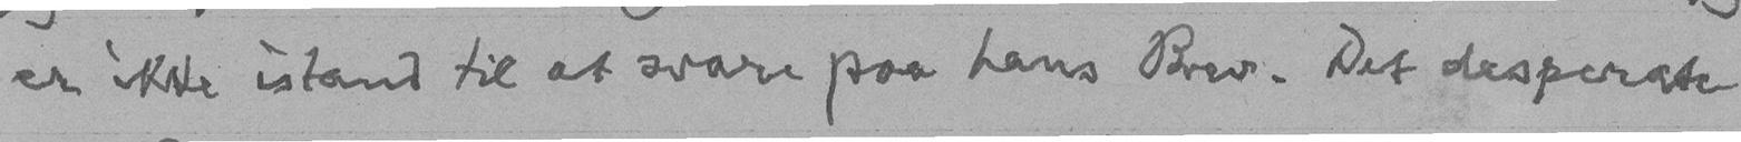er ikke istand til at svare paa hans Brev. Det desperate
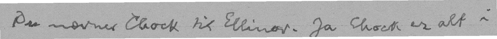Du nævner Chock til Ellinor. Ja Chock er alt i
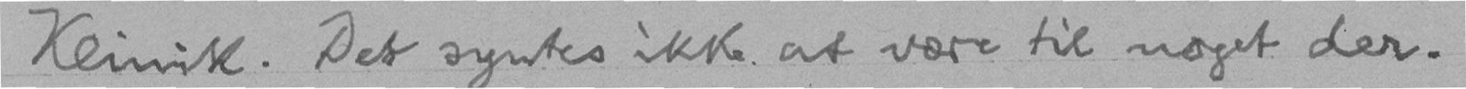Klinik. Det syntes ikke at være til noget der.
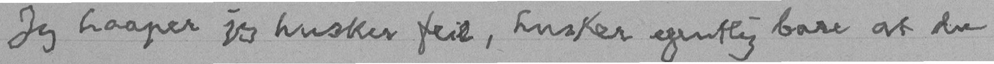Jeg haaper jeg husker feil, husker egentlig bare at du
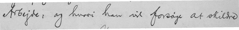Arbejde, og hvori han vil forsøge at skildre
